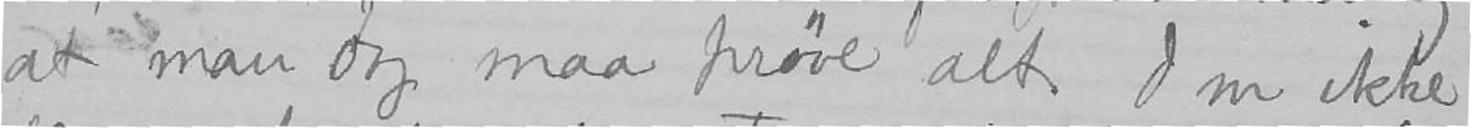at man dog maa prøve alt. Om ikke
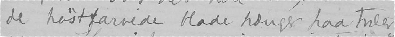de høstfarvede blade hænger paa trær-
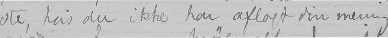ste, hvis du ikke har aflagt din mening
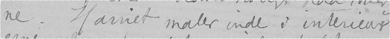ne. Harriet maler inde i interieur-
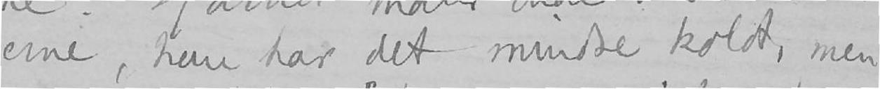erne, hun har det mindre koldt, men
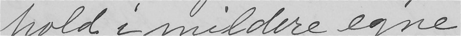hold i mildere egne
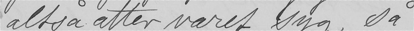altså atter været syg, så
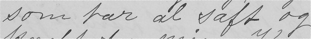som tar al saft og
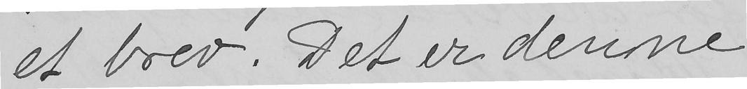et brev. Det er denne
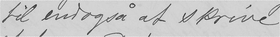til endogså at skrive
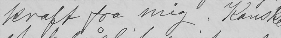kraft fra mig. Kanske
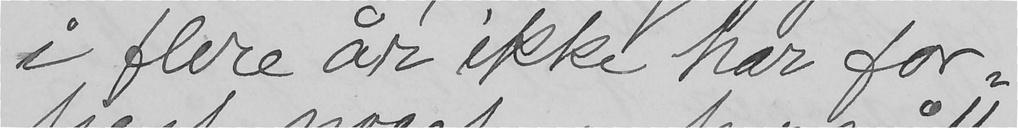i flere år ikke har for-
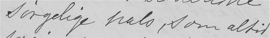sørgelige hals, som altid
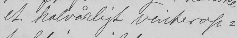et halvårligt vinterop-
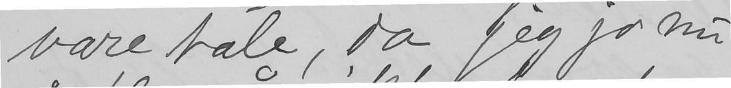være tale, da jeg jo nu
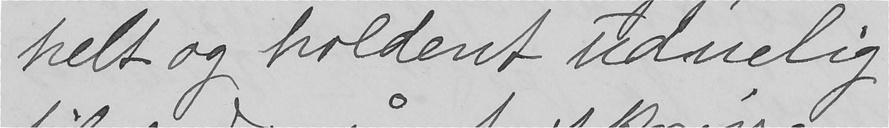helt og holdent uduelig
